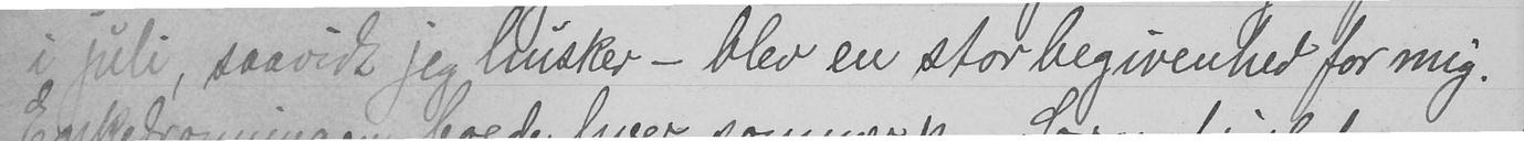i juli, saavidt jeg husker - blev en stor begivenhed for mig.
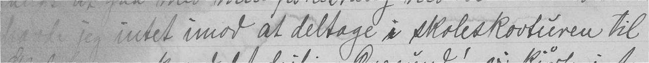hadde jeg intet imod at deltage i skoleskovturen til
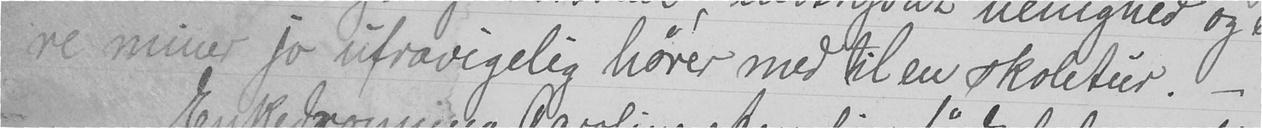re miner jo ufravigelig hører med til en skoletur. -
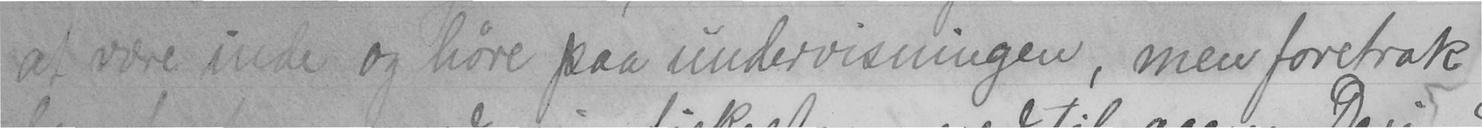at være inde og høre paa undervisningen, men foretrak
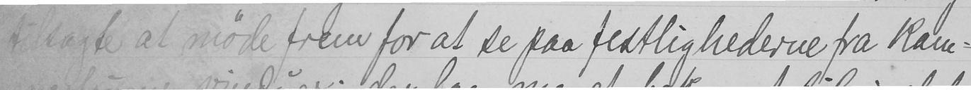tilsagte at møde frem for at se paa festlighederne fra kam-
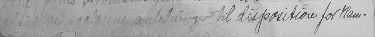altid ved saadanne anledninger til disposition for kam-
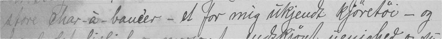store char-à-banc'er - et for mig ukjendt kjøretøi - og
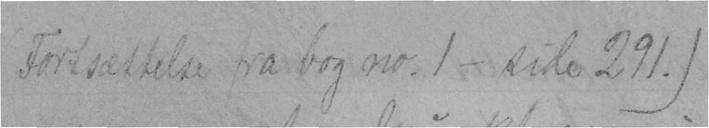Fortsættelse fra bog. no. 1 - side 291.)
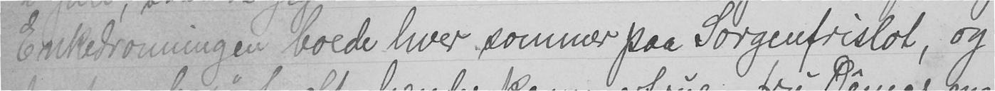Enkedronningen boede hver sommer paa Sorgenfrislot, og
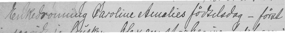Enkedronning Caroline Amalies fødselsdag - først
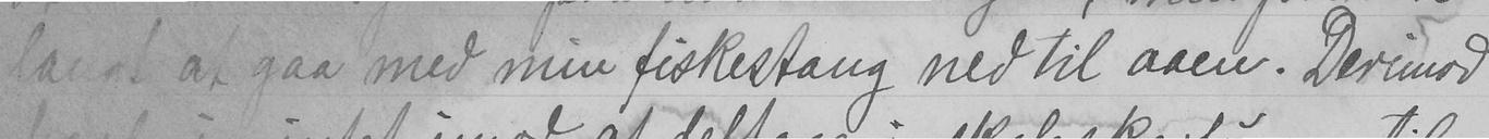langt at gaa med min fiskestang ned til aaen. Derimod
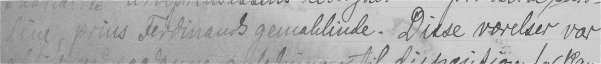line, prins Ferdinands gemahlinde. Disse værelser var
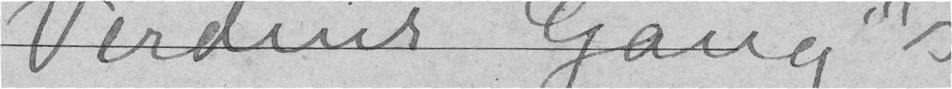Verdens Gang’s
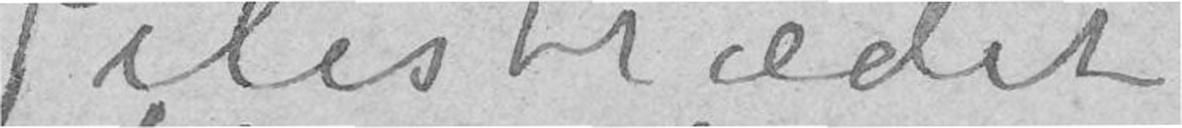Pilestrædet
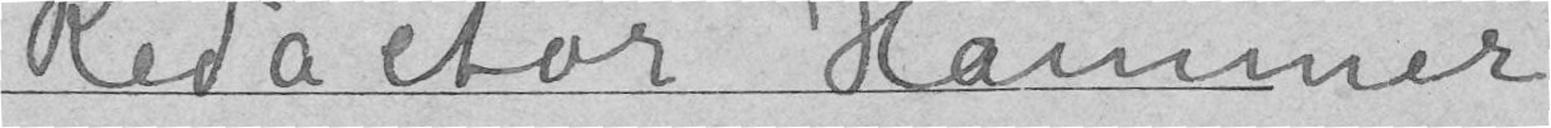M Redactør Hammer
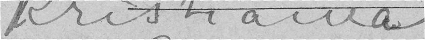Kristiania
